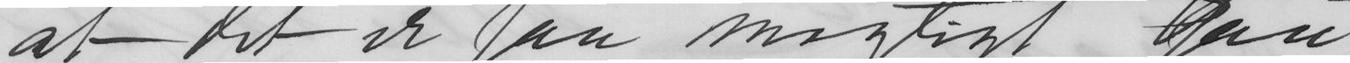at det er saa mægtigt saa
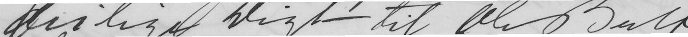deilige Digt til Ole Bull
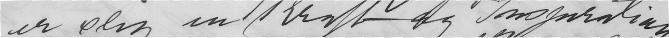er slig en Kraft og Inspiration
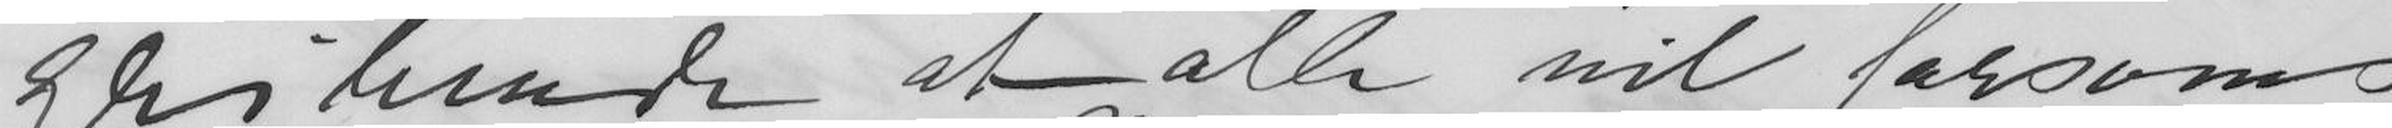glimrende at alle vil forsones
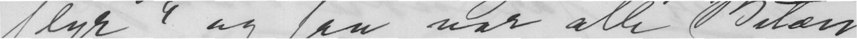flyr og saa var alle Betæn-
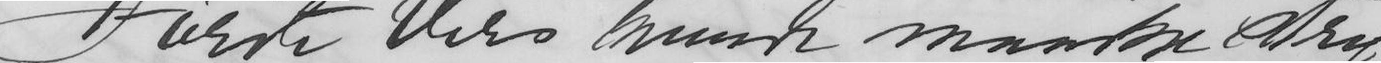Første Vers kunde maaske stry-
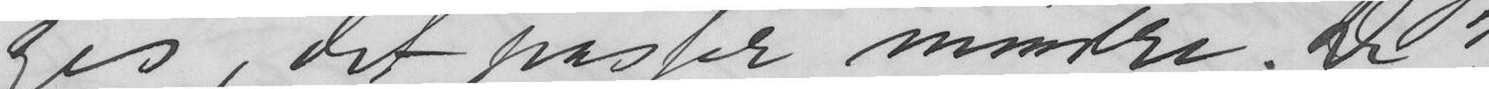ges, det passer mindre. De 3
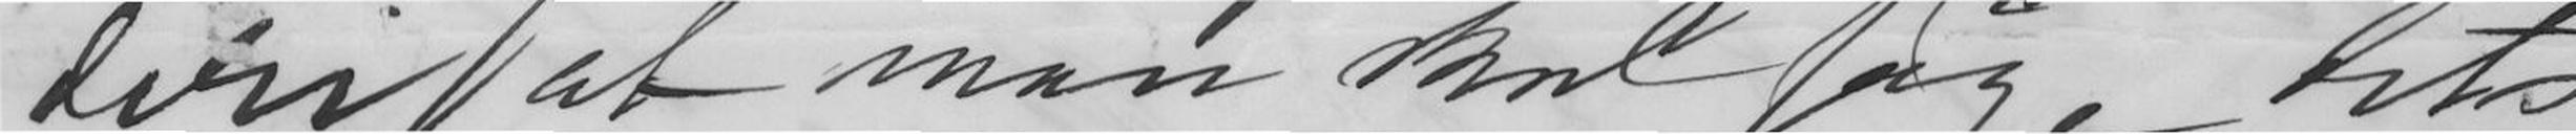deri at man skal søge dets
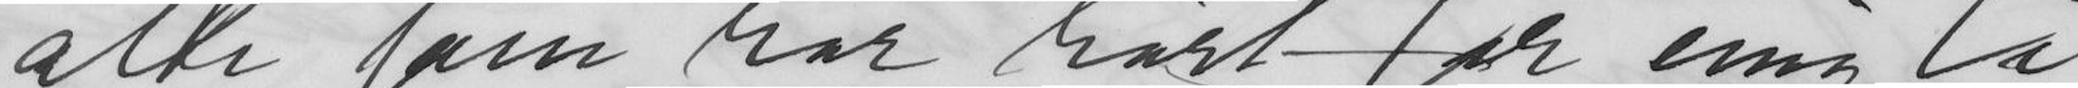alle som har hørt er enig i
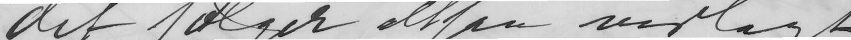det følger altsaa vedlagt.
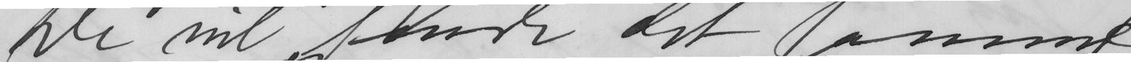De vil finde det samme
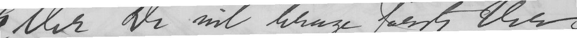Eller De vil bruge første Vers
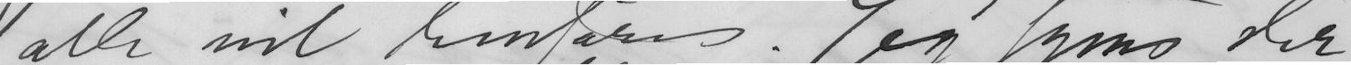alle vil henføres: Jeg synes der
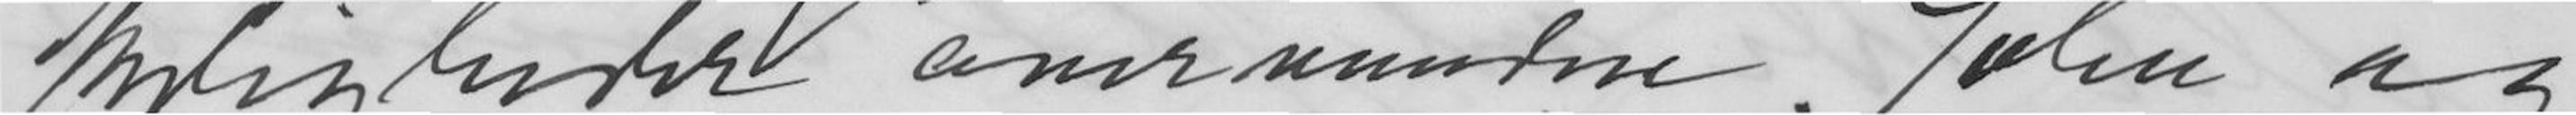keligheder overvundne. John og
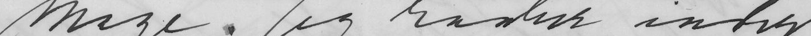mage. Jeg haaber inderlig
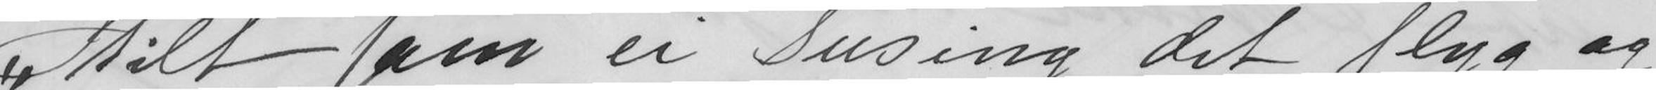Stilt som en susing det flyg og
